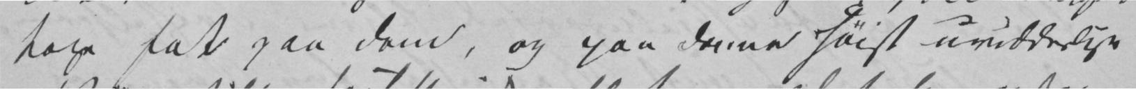tage fat paa dem, og paa denne høist uridderlige
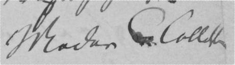Moder C. Collett
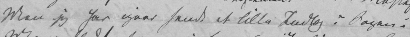Men jeg har igaar sendt et lille Indlæg i Sagen i
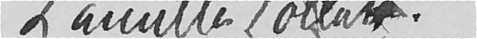Camilla Collett.
